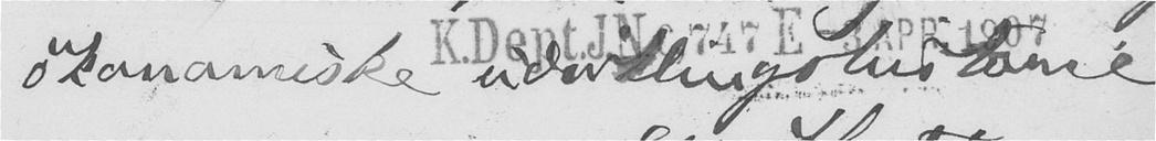økonomiske udviklingshistorie.
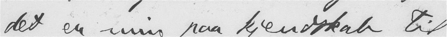det er min paa kjendskab til
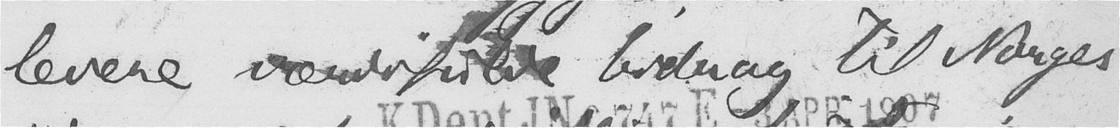levere værdifulde bidrag til Norges
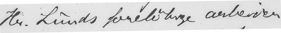Hr. Lunds foreløbige arbeider
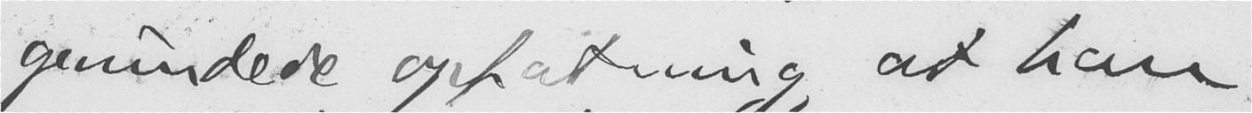grundede opfatning, at han
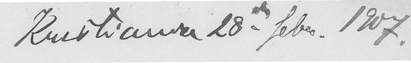Kristiania 28de febr. 1907.
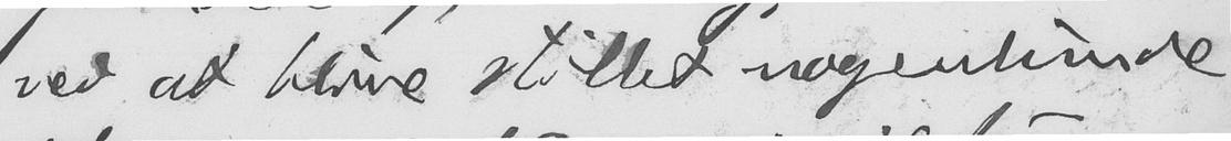ved at blive stillet nogenlunde
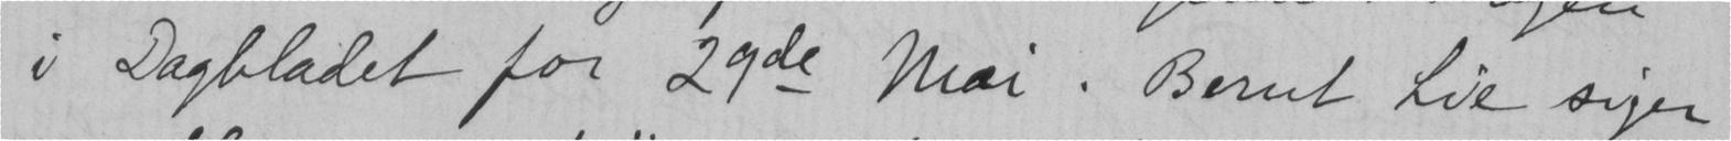i Dagbladet for 29de mai. Berut Lie siger
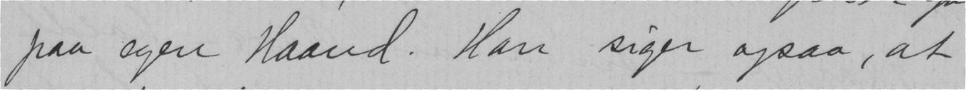paa egen Haand. Han siger ogsaa, at
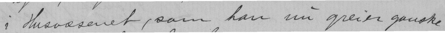i Husvæsenet, som han nu greier ganske
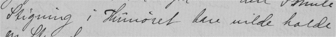Stigning i Humøret bare vilde holde
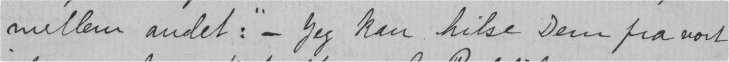mellem andet: " - Jeg kan hilse Dem fra vort
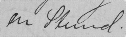en Stund.
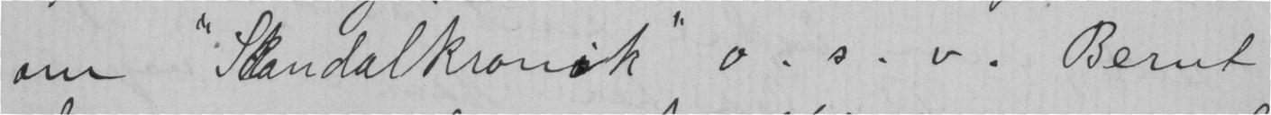om "Skandalkronik" o. s. v. Berut
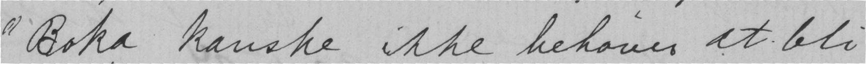"Boka kanske ikke behøver at bli
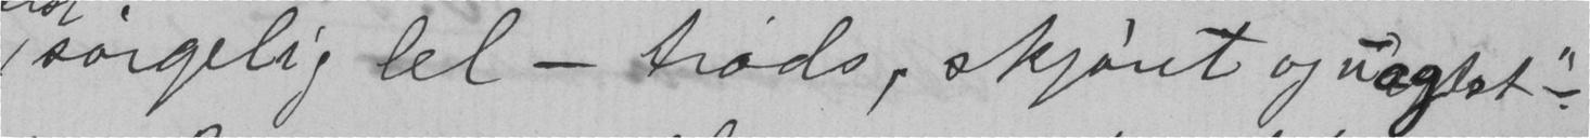sørgelig lel - trods, skjønt og uagtet - "
